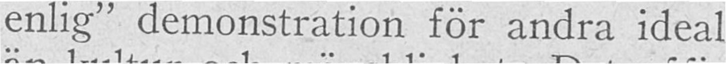enlig" demonstration för andra ideal
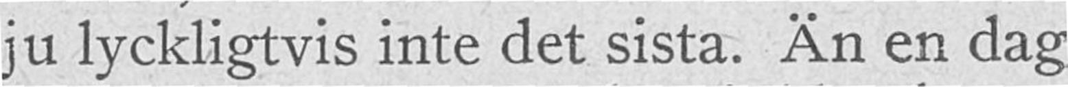ju lyckligtvis inte det sista. Än en dag
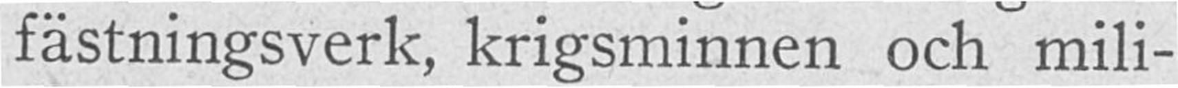fästningsverk, krigsminnen och mili-
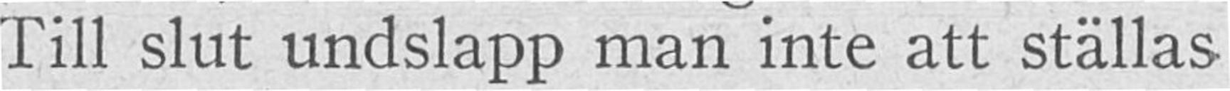Till slut undslapp man inte att ställas
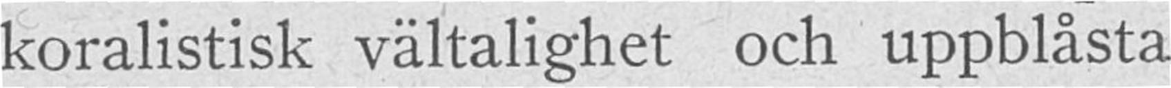koralistisk vältalighet och uppblåsta
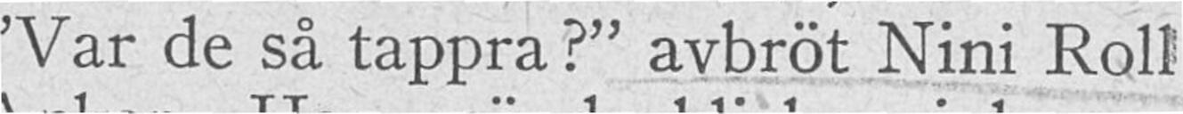"Var de så tappra?" avbröt Nini Roll
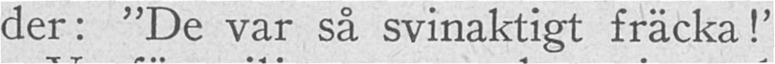der: "De var så svinaktigt fräcka!"
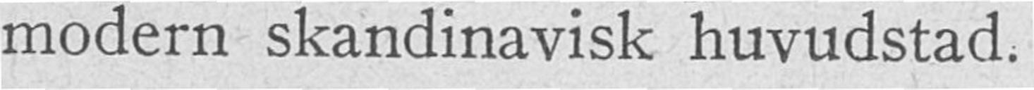modern skandinavisk huvudstad.
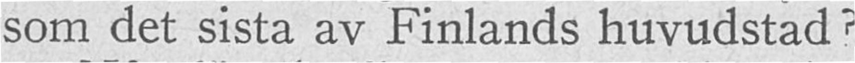som det sista av Finlands huvudstad?
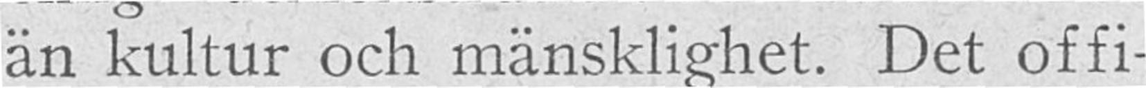än kultur och mänsklighet. Det offi-
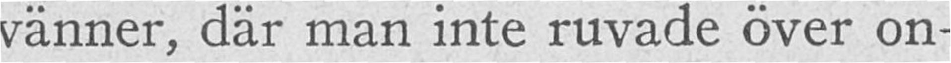vänner, där man inte ruvade över on-
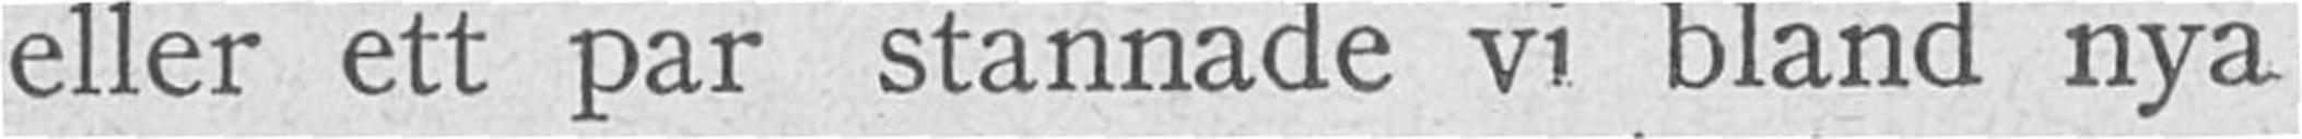eller ett par stannade vi bland nya
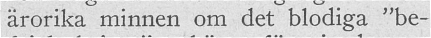ärorika minnen om det blodiga "be-
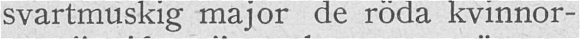svartmuskig major de röda kvinnor-
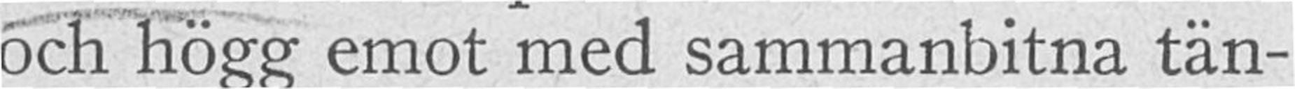och hogg emot med sammanbitna tän-
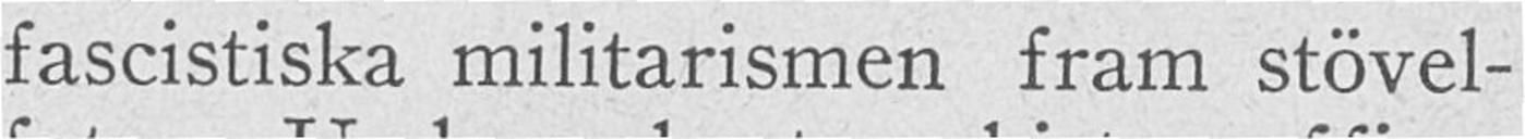fascistiska militarismen fram stövel-
